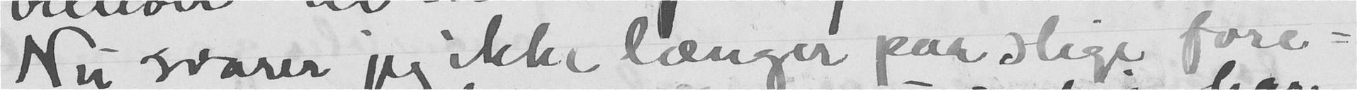Nu svarer jeg ikke længer paa slige fore-
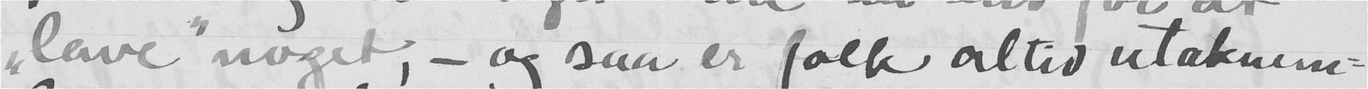"lave" noget, - og saa er folk altid utaknem-
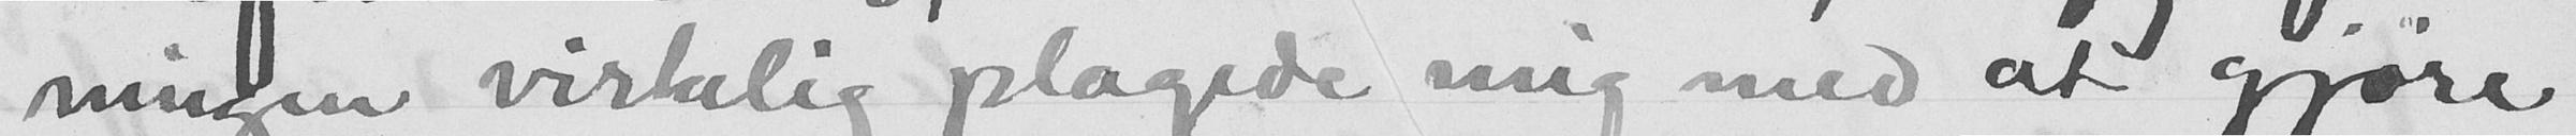ningen virkelig plagede mig med at gjøre
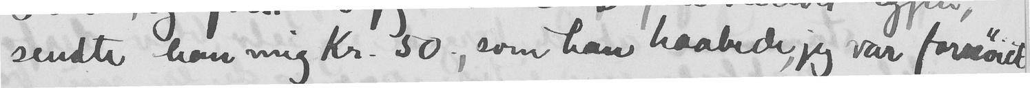sendte han mig Kr. 50, som han haabede, jeg var fornøiet
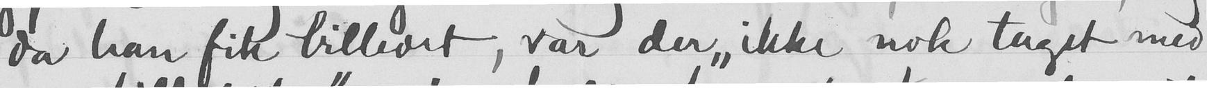da han fik billedet, var der "ikke nok taget med
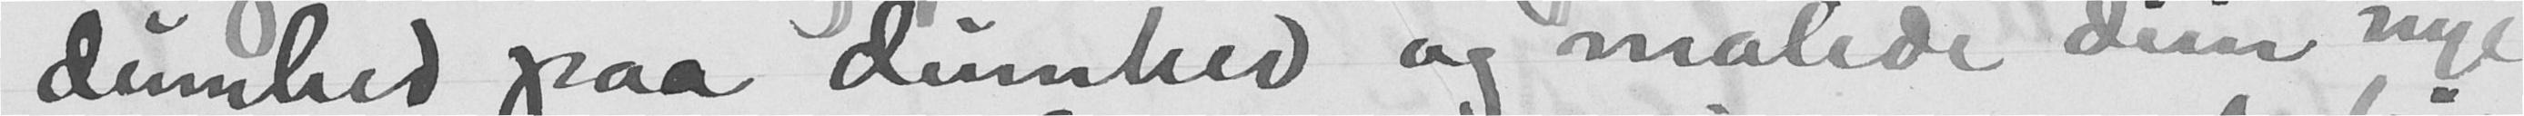dumhed paa dumhed og malede dem nye
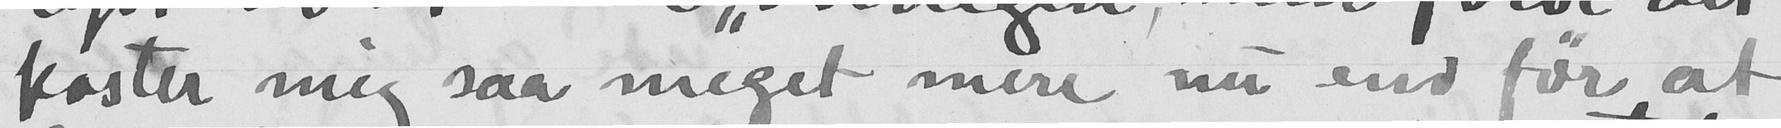koster mig saa meget mere nu end før at
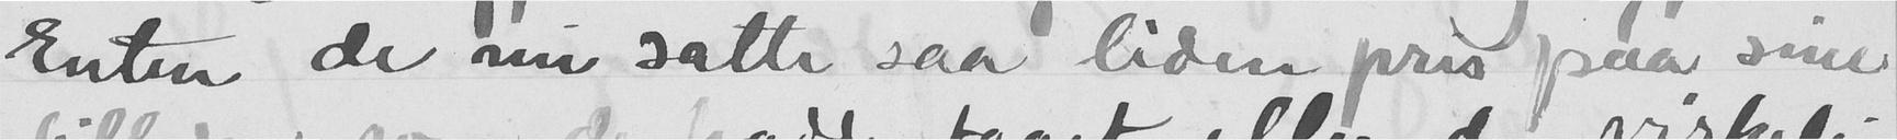Enten de nu satte saa liden pris paa sine
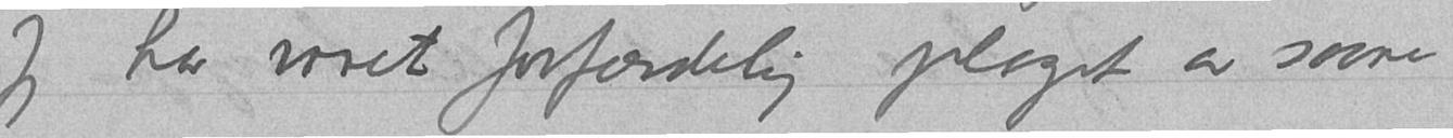Jeg har været forfærdelig plaget av saare
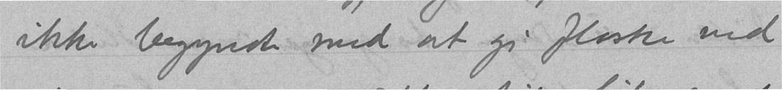ikke begyndte med at gi flaske ved
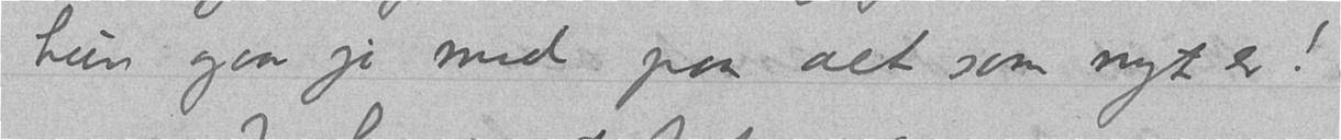hun gaar jo med paa alt som nyt er!
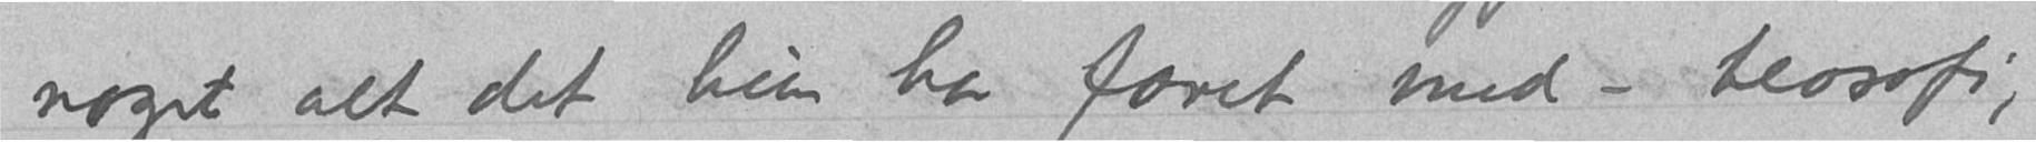noget alt det hun har faret med - teosofi,
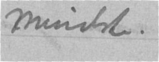mindste.
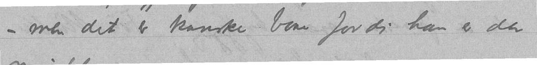- men det er kanske bare fordi han er den
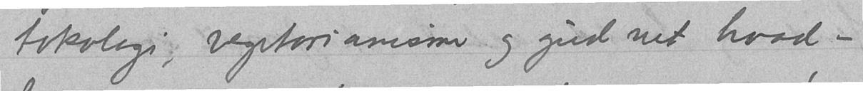tokologi, vegitarianisme og gud vet hvad-
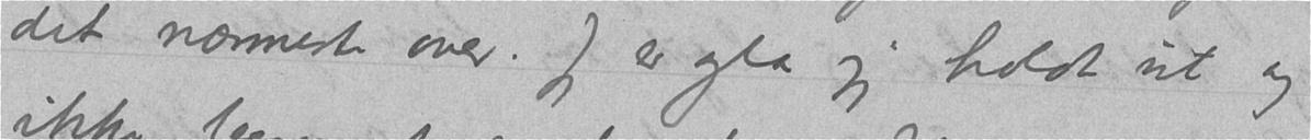det nærmeste over. Jeg er gla jeg holdt ut og
